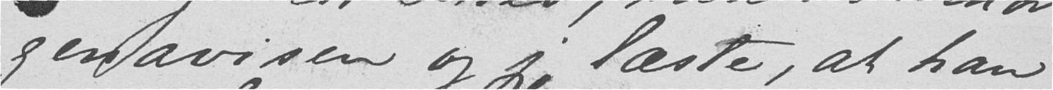genavisen og jeg læste, at han
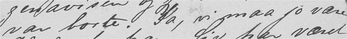var borte. Ja, vi maa jo være
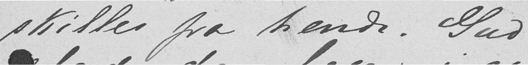skilles fra hende. Gud
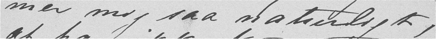mer mig saa naturligt,
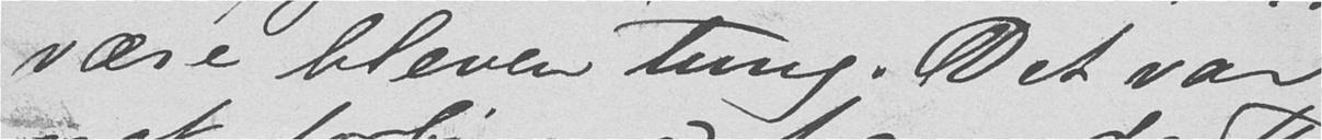være bleven tung. Det var
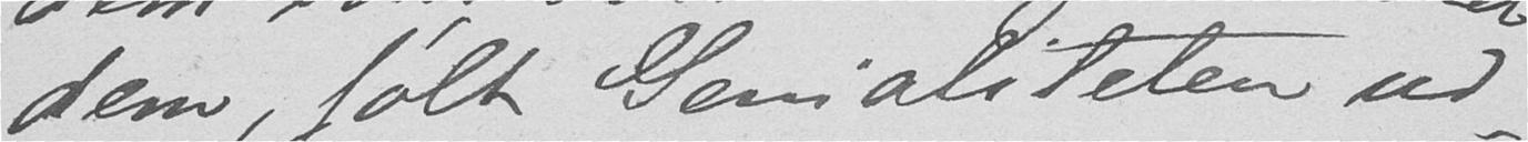dem, følt Genialiteten ud-
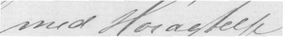med Høiagtelse
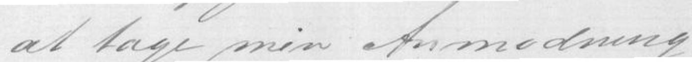at tage min Anmodning
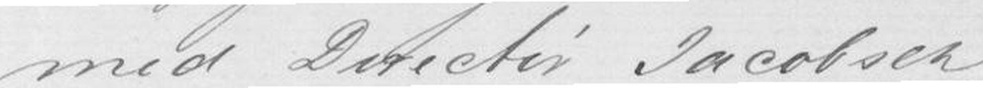med Derectør Jacobsen
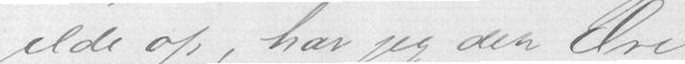ilde op, har jeg den Ære
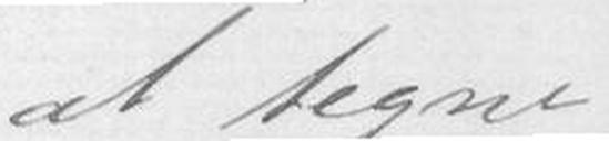at tegne
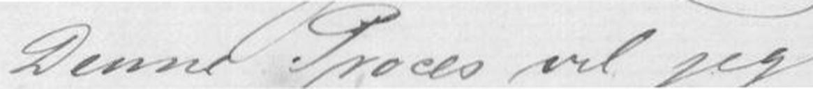Denne Proces vil jeg
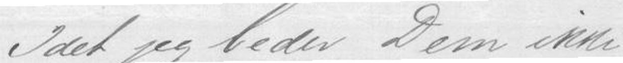Idet jeg beder Dem ikke
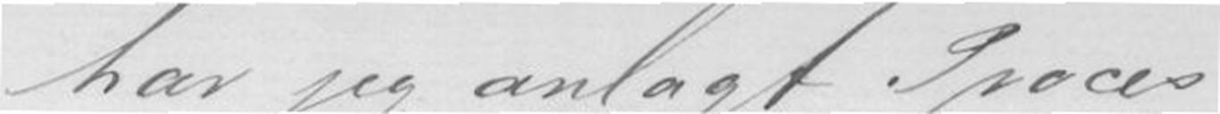har jeg anlagt Proces
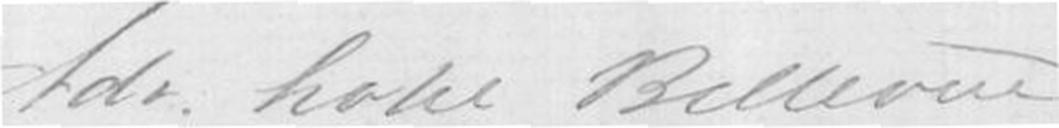Adr. hotel Bellevue
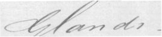Glands.
